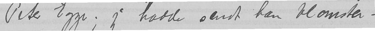Peter Egge; jeg hadde sendt han blomster -
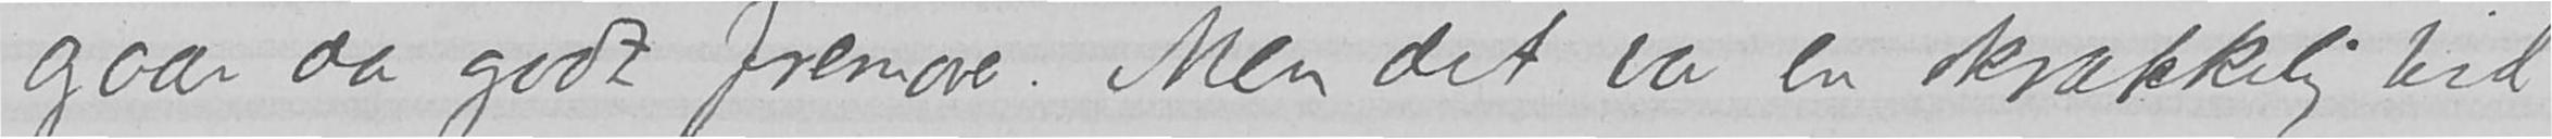gaar da godt fremover. Men det var en skrækkelig tid
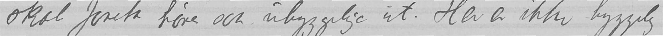skal foreta høres saa uhyggelig ut. Her er ikke hyggelig
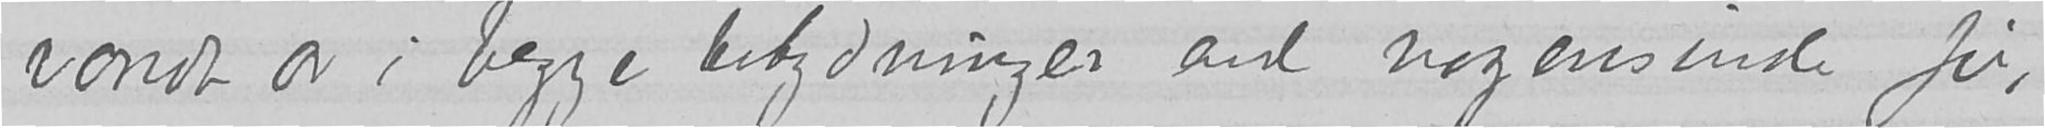vondt av i begge betydninger end nogensinde før,
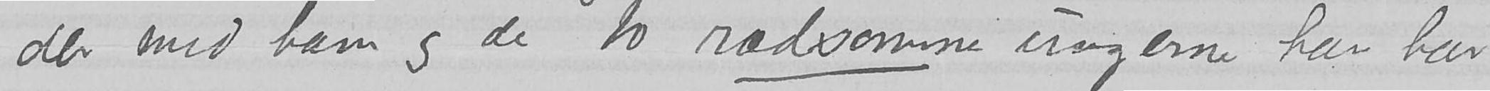der med ham og de to rædsomme ungerne han har
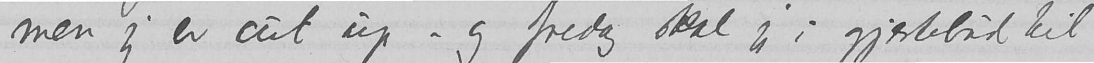men jeg er cut up – og fredag skal jeg i gjestebud til
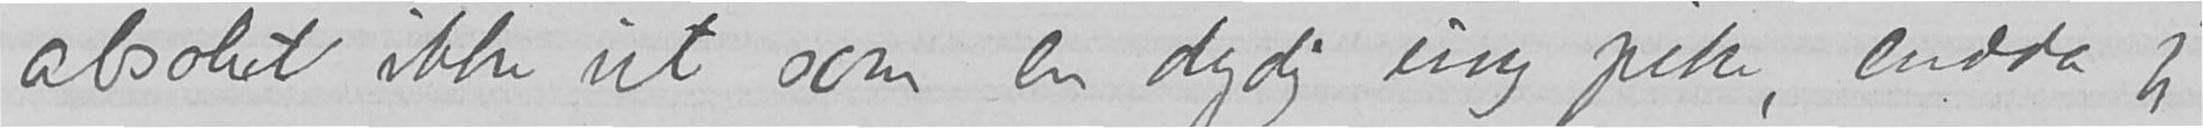absolut ikke ut som en dydig ung pike, endda jeg
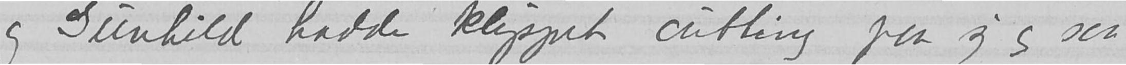og Gunhild hadde klippet cutting paa sig og saa
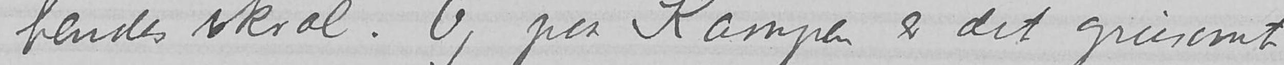hendes skral. Og paa Kampen er det grusomt –
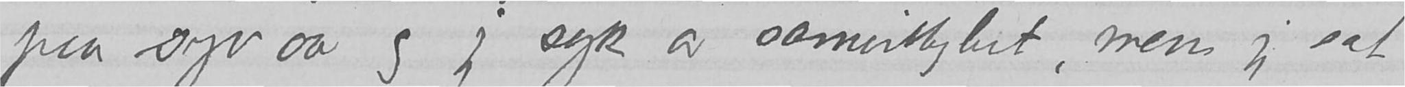paa syv aar og jeg syk av samvittighet, mens jeg sat
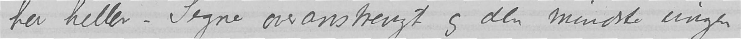her heller. Signe overanstrengt og den mindste ungen
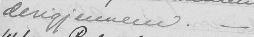derigjennem. -
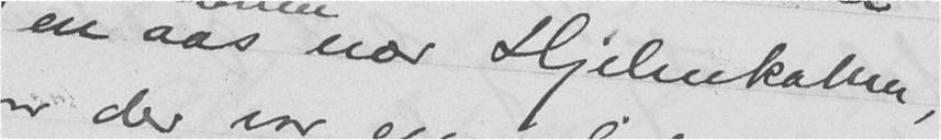en aas nær Hjelmkollen,
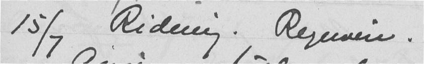15/7 Ridning. Regnveir.
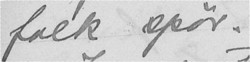folk spør.
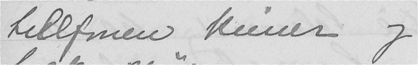telefonen kimer og
